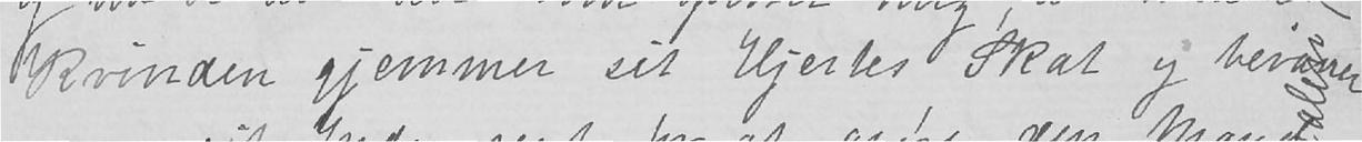Kvinden gjemmer sit Hjertes Skat og bevarer
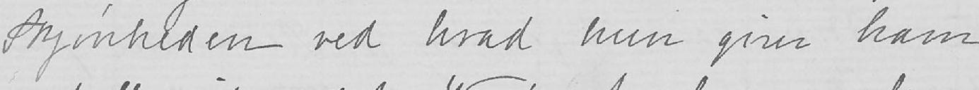Skjønheden ved hvad hun giver ham
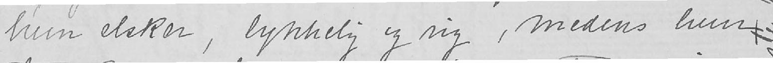hun elsker, lykkelig og rig, medens hun
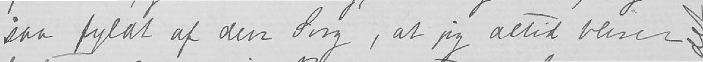saa fyldt af den Sorg, at jeg altid bliver
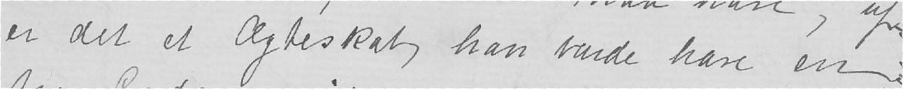er det et Ægteskab, han burde have en
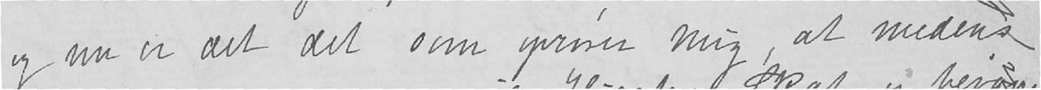og nu er det det som oprører mig, at medens
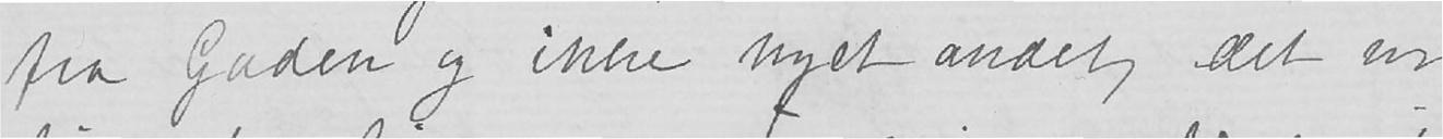fra Gaden og ikke noget andet, det er
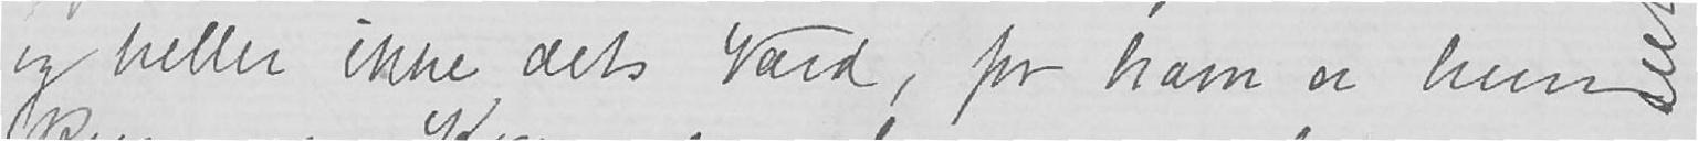og heller ikke dets Værd, for ham er hun
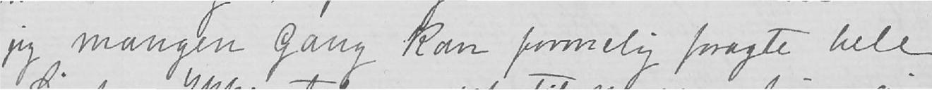jeg mangen Gang kan formelig foragte hele
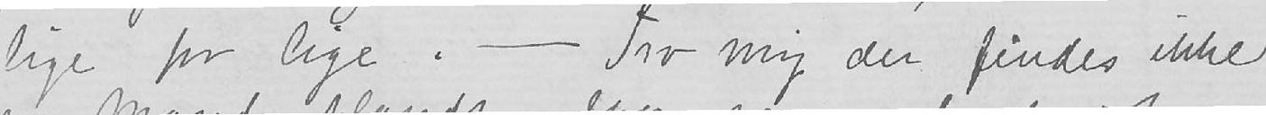lige for lige. – Tro mig der findes ikke
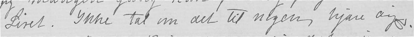Livet. Ikke tal om det til nogen, kjære dig,
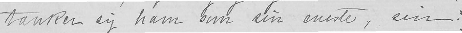tænker sig ham som sin eneste, sin
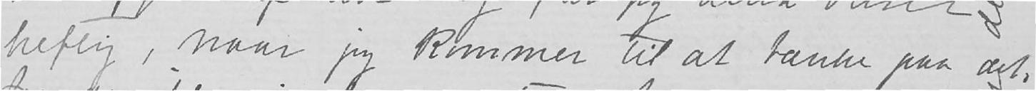heftig, naar jeg kommer til at tænke paa det.
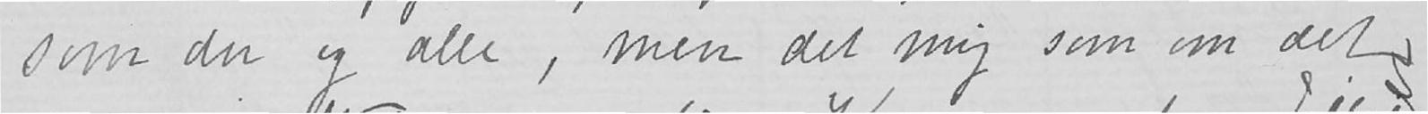som du og alle, men det mig som om det
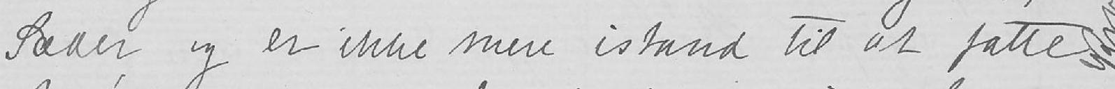Sæder og er ikke mere istand til at fatte
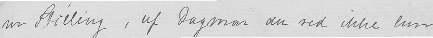vor Stilling, uf Dagmar du ved ikke hvor
